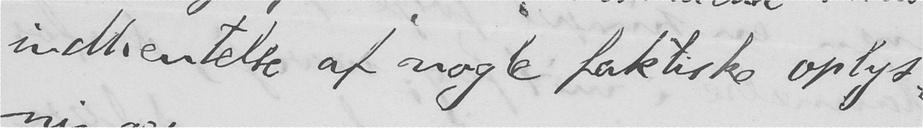indhentelse af nogle faktiske oplys-
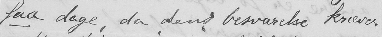faa dage, da dens besvarelse kræver
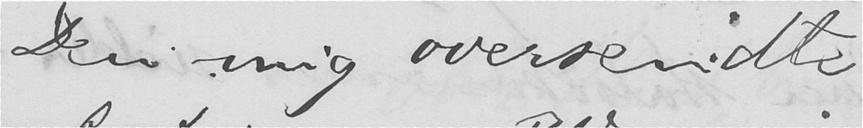Den mig oversendte
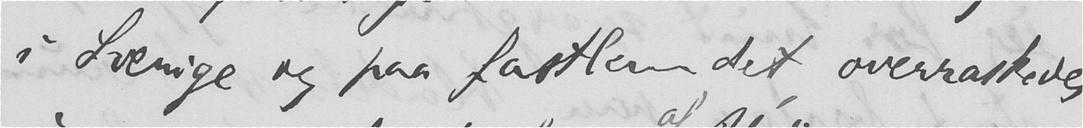i Sverige og paa fastlandet, overraskedes
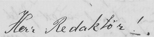Herr Redaktør!
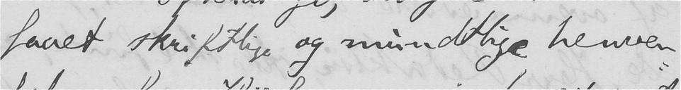faaet skriftlige og mundtlige henven-
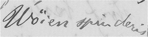Wøien spinderis
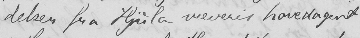delser fra Hjula væveris hovedagent
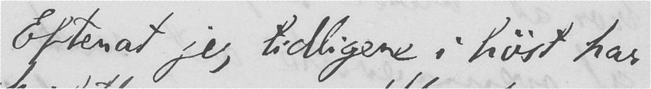Efterat jeg tidligere i høst har
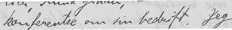konferentse om sin bedrift. Jeg
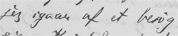jeg igaar af et besøg
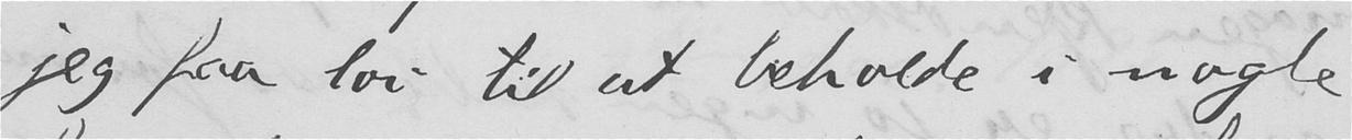jeg faa lov til at beholde i nogle
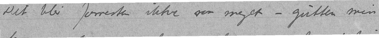Det blir forresten ikke saa meget – gutten min
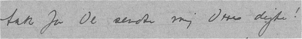tak for De sendte mig Deres digte!
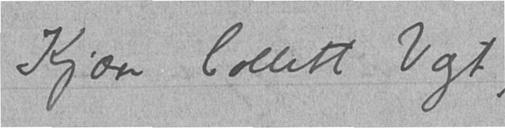Kjære Collett Vogt,
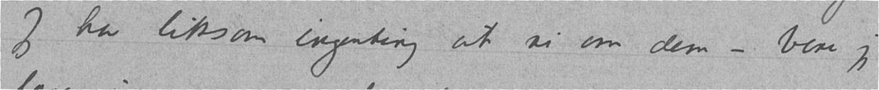Jeg har liksom ingenting at si om dem – bare jeg
